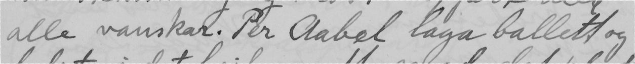alle vanskar. Per Aabel laga ballett og
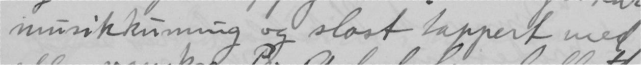musikkunning og slost tappert med
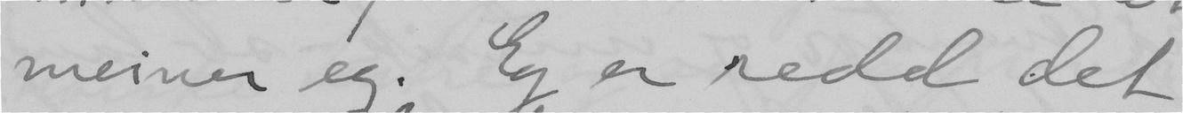meiner eg. Eg er redd det
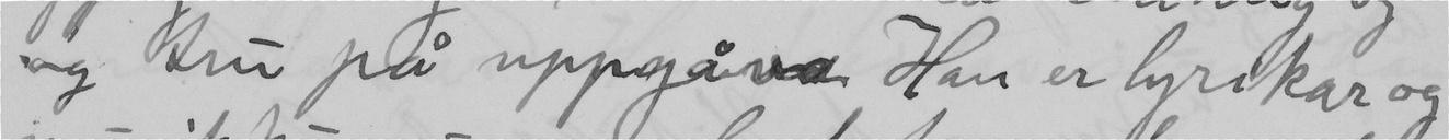og tru på uppgåva. Han er lyrikar og
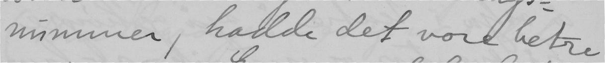nummer, hadde det vore betre
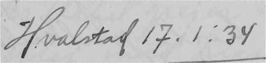Hvalstad 17.1.34
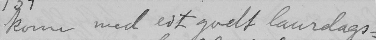kome med eit godt laurdags-
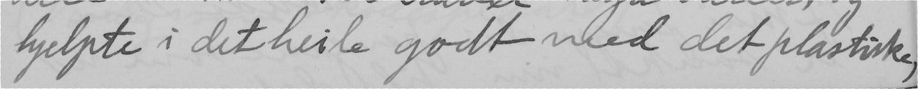hjelpte i det heile godt med det plastiske,
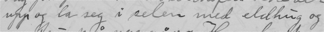upp og la seg i selen med eldhug og
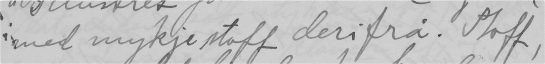med mykje stoff derifra. Stoff,
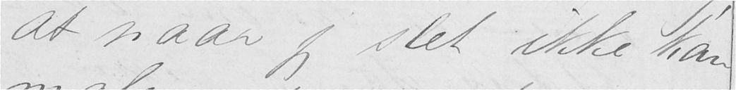at naar jeg slet ikke kan
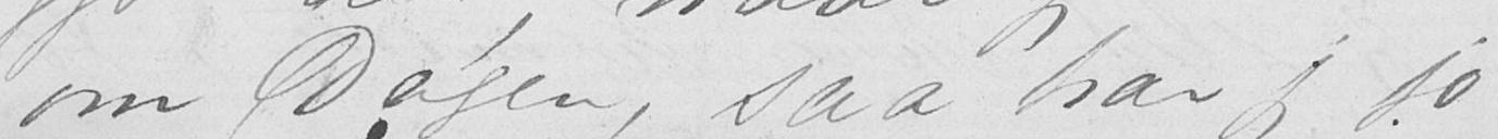om Dagen, saa har jeg jo
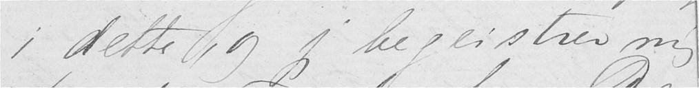i dette, og jeg begeistrer mig
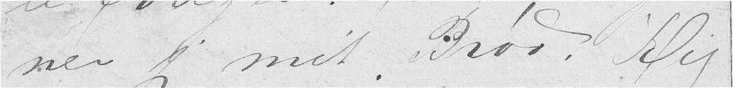ner jeg mit Brød. Rig-
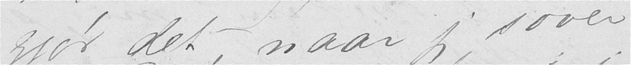gjør det naar jeg sover
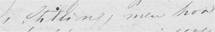i Stilling; men hvad
